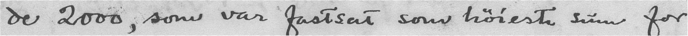de 2000, som var fastsat som høieste sum for
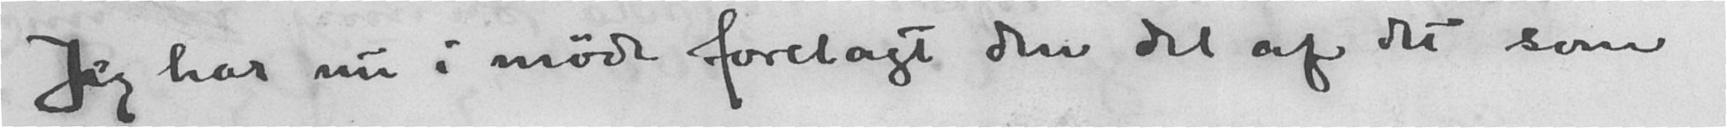Jeg har nu i møde forelagt den del af det som
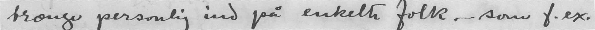trænge personlig ind på enkelte folk — som f.ex.
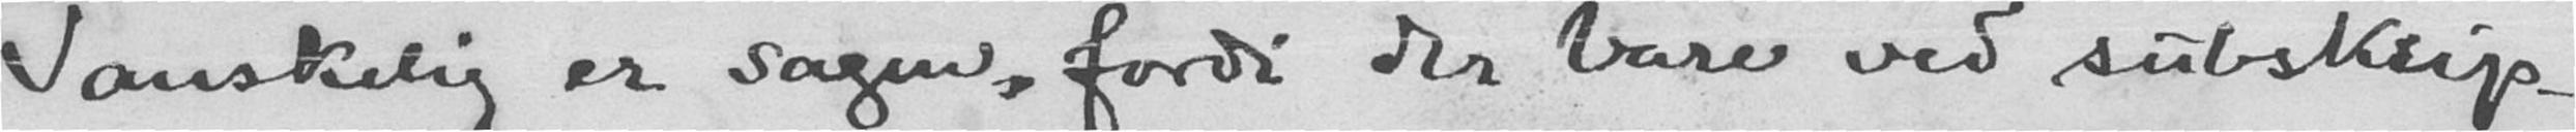Vanskelig er sagen, fordi der bare ved subskrip-
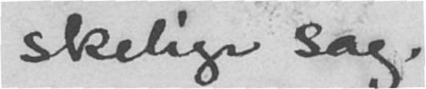skelige sag.
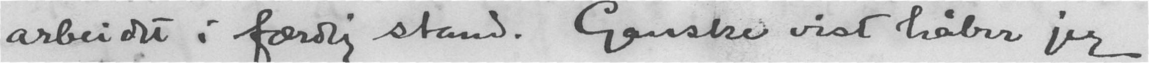arbeidet i færdig stand. Ganske vist håber jeg
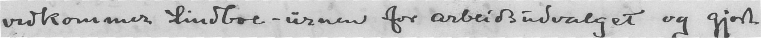vedkommer Lindboe-urnen for arbeids-udvalget og gjort
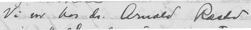Vi var hos dr. Arnold Ræstad
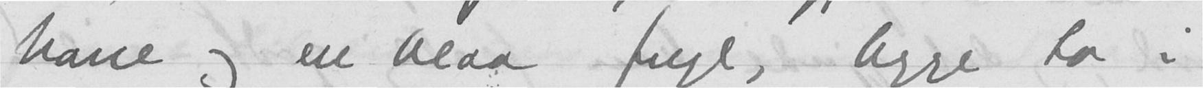hane og en blaa fugl, begge to i
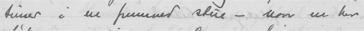timer i en fremmed stue - naar en har
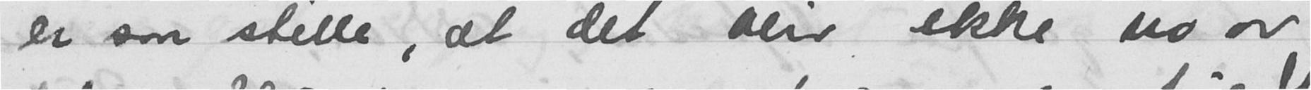er saa stille, at det blir ikke no av
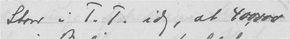Staar i T. T. idag, at 400,000
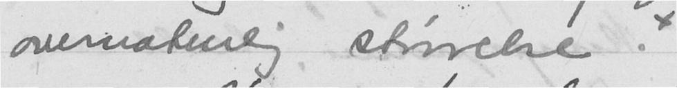overnaturlig størrelse.*
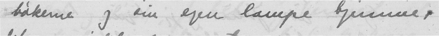bøkerne og sin egen lampe hjemme,
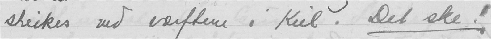streikes ved værftene i Kiel. Det ske!
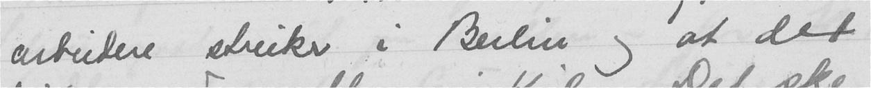arbeidere streiker i Berlin og at det
